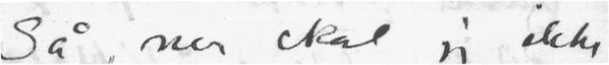Så ner skal jeg ikke
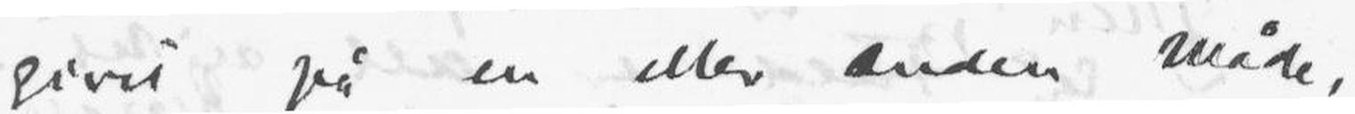givet på en eller Andet måde,
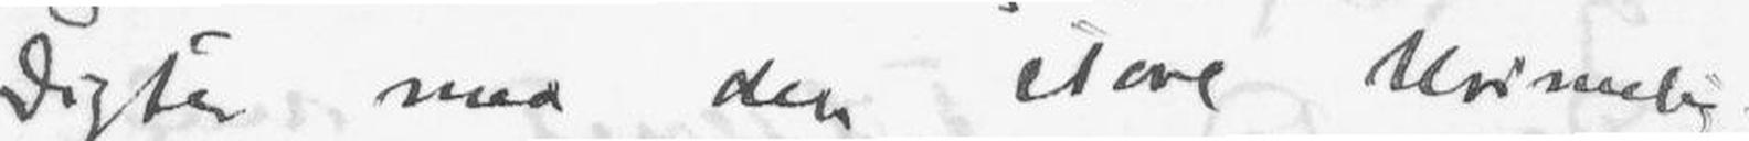Digter med den store Urimelig-
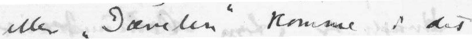eller Dævelen komme i det
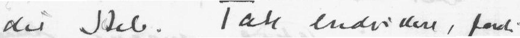det Hele. Tak endvidere, fordi
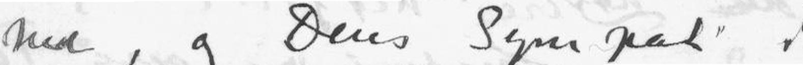hed, og Deres Sympati i
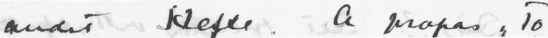andet Hefte. A propos To
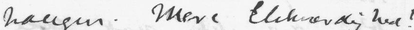haugen. Mere Elskværdighed!
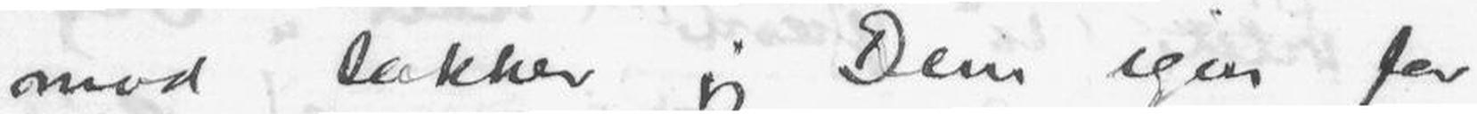mod takker jeg Dem igen for
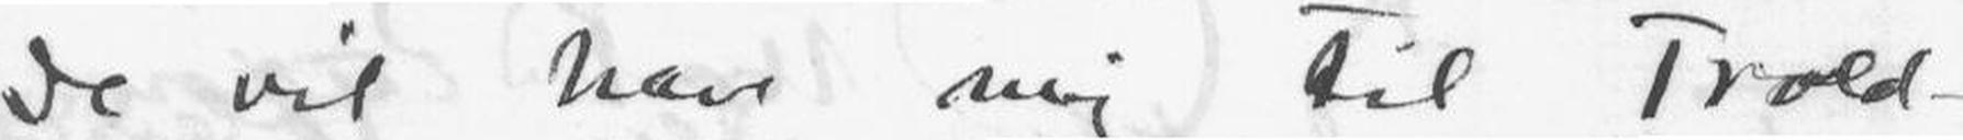De vil have mig til Trold-
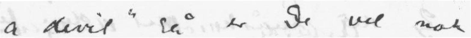a devil så er De vel nok
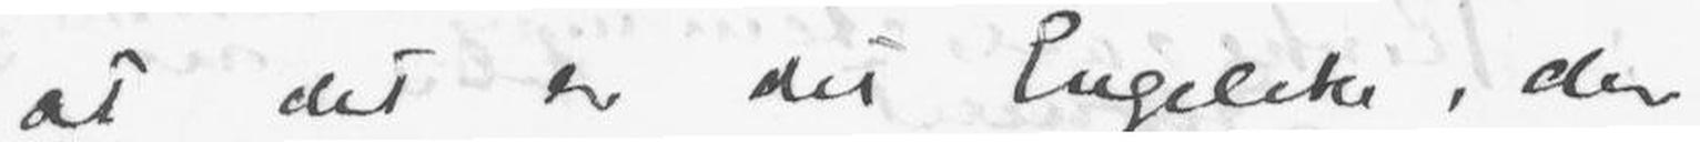at det er det Engelske, der
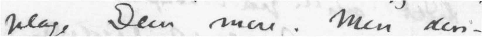plage Dem mere. Men deri-
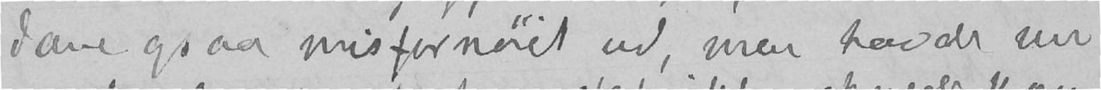Jane ogsaa misfornøiet ud, men havde nu
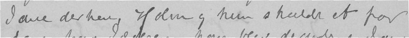Jane derhen, Holm og hun skulde et par
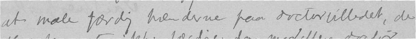at male færdig mænderne paa doctorbilledet, de
